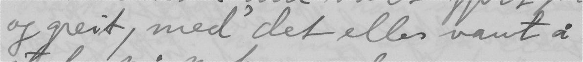og greit, med det elles vant å
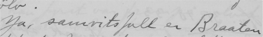Ja, samvitsfull er Braaten
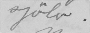sjölv.
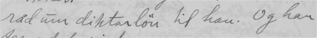råd um diktarlön til han. Og han
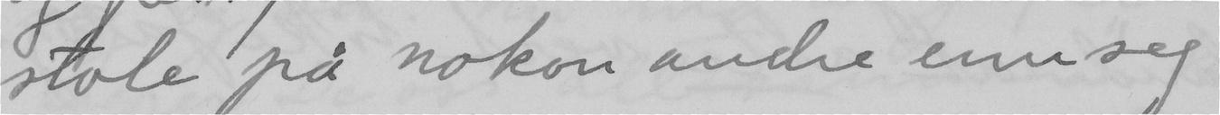stole på nokon andre enn seg
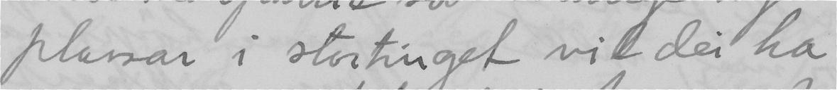plassar i stortinget vil dei ha
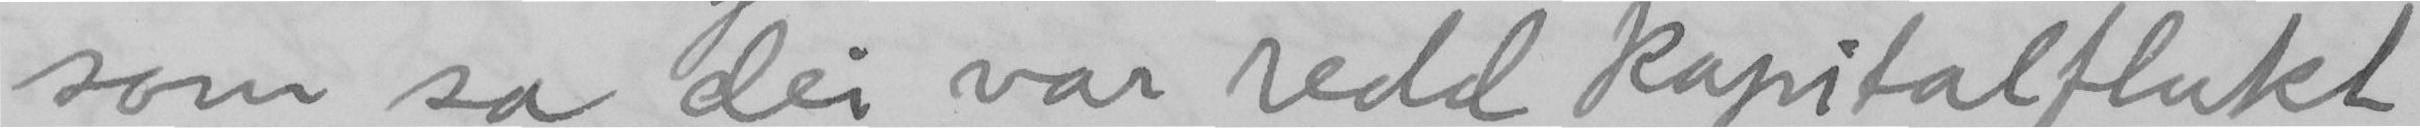som sa dei var redd kapitalflukt
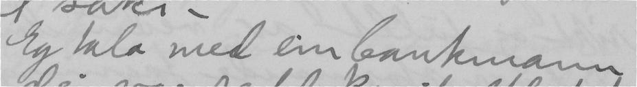Eg tala med ein bankmann
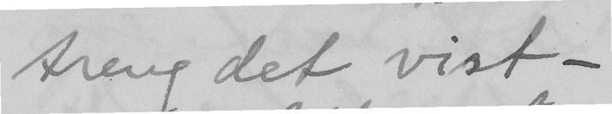treng det vist –
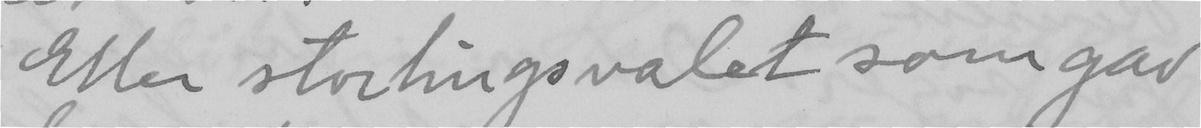Etter stortingsvalet som gav
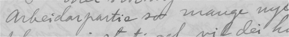arbeidarpartie so mange nye
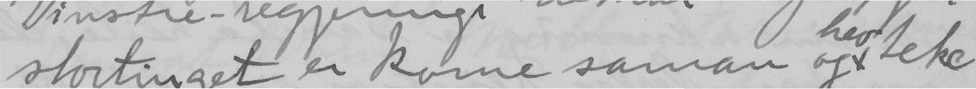stortinget er kome saman og heve teke
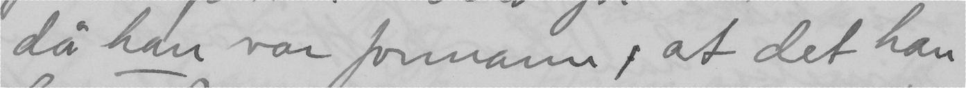då han var formann, at det han
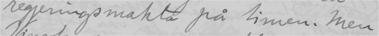regjeringsmakta på timen. Men
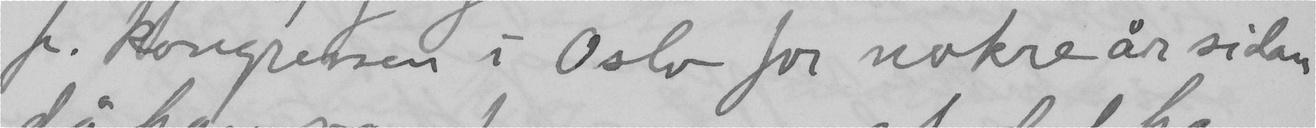f. kongressen i Oslo for nokre år sidan
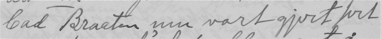bad Braaten um vart gjort fort
